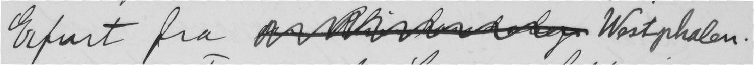Erfurt fra  Westphalen.
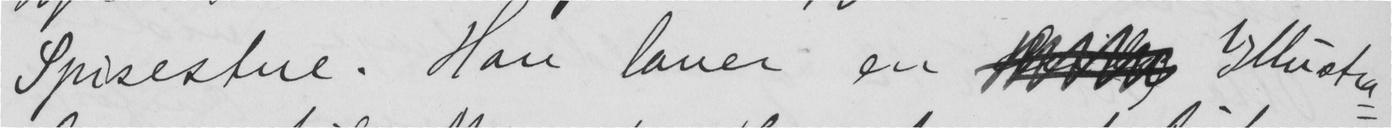Spisestue. Han laver en  Illustra-
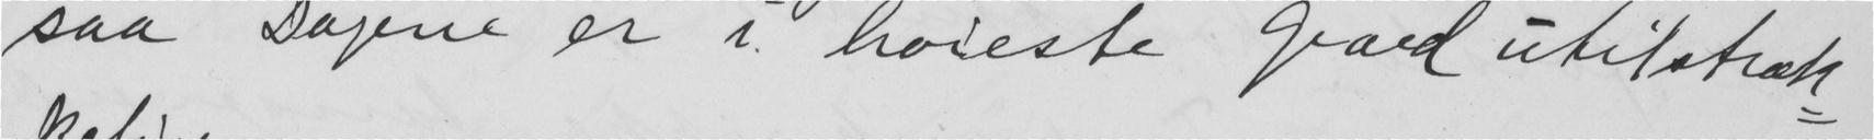saa Dagene er i høieste grad utilstræk-
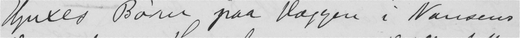Hjuxes Børn, paa Væggen i Nansens
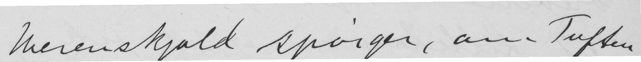Werenskjold spørger, om Tuften
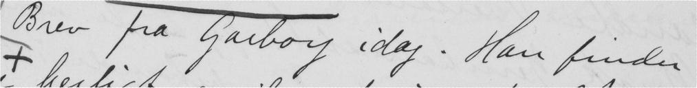Brev fra Garborg idag. Han finder
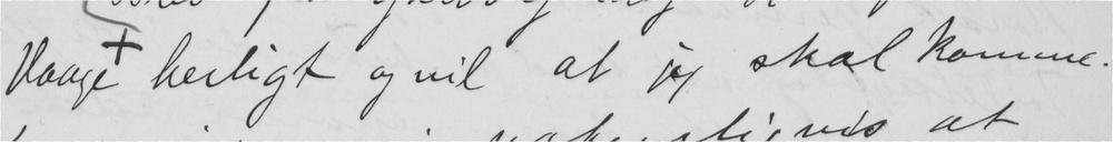Vaage herligt og vil at jeg skal komme.
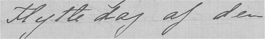Flyttedag af den
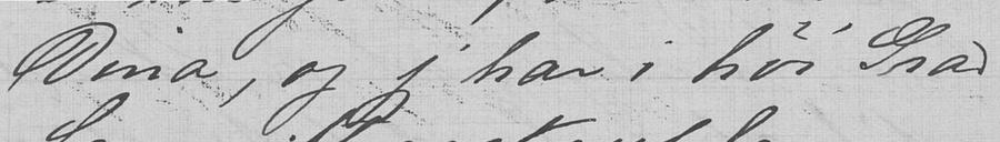Dina, og jeg har i høi Grad
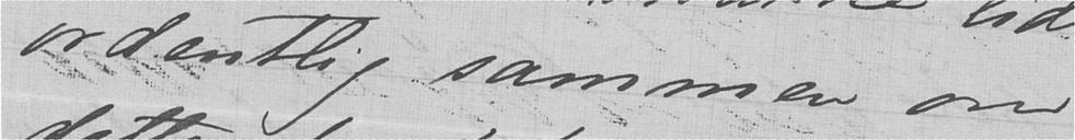ordentlig sammen om
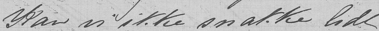Kan vi ikke snakke lidt
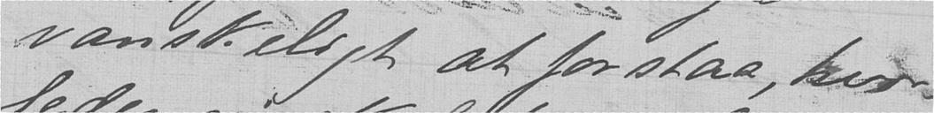vanskeligt at forstaa, hvor-
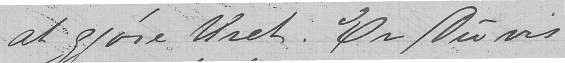at gjøre Uret. Er Du vis
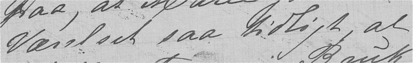Værelset saa tidligt at
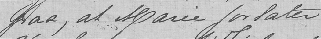paa, at Marie forlater
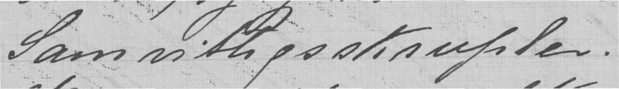Samvittigsskrupler.
